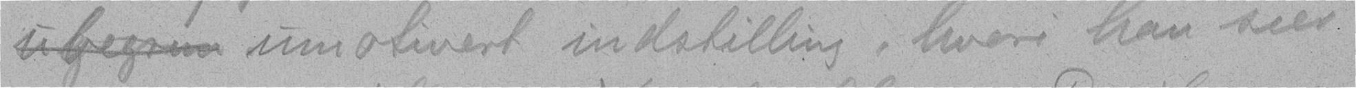ubegrun umotivert indstilling, hvori han sier
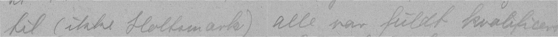til (ikke Holtsmark) alle var fuldt kvalificert
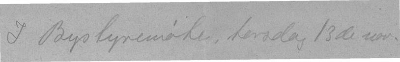I Bystyremøte, torsdag 13de nov.
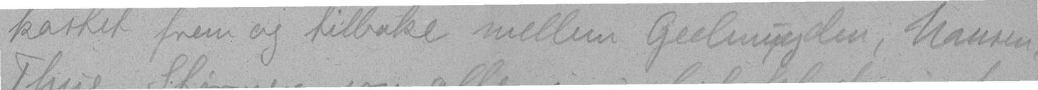kastet frem og tilbake mellem Geelmuyden, Nansen,
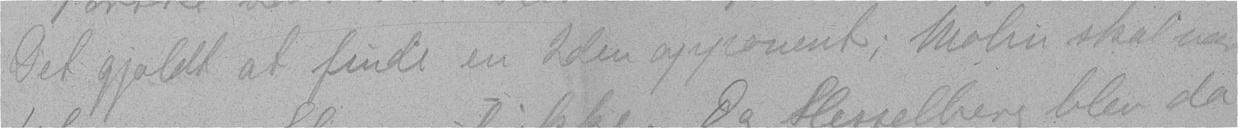Det gjaldt at finde en 2den opponent; Mohn skal være
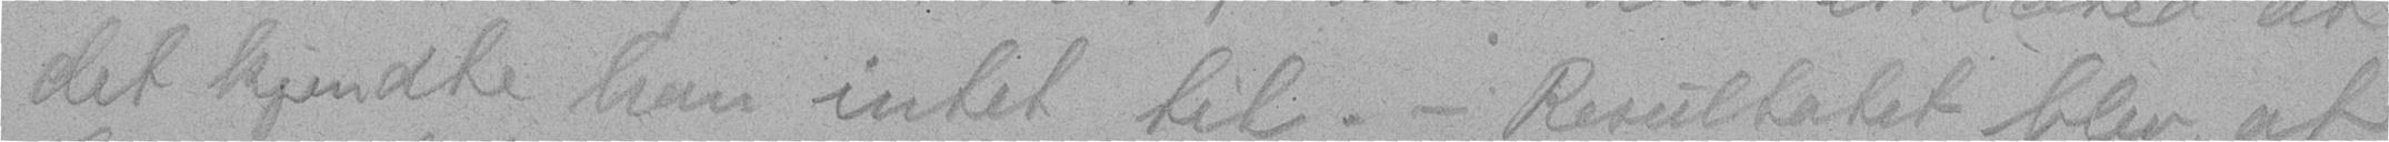det kjendte han intet til. - Resultatet blev, at
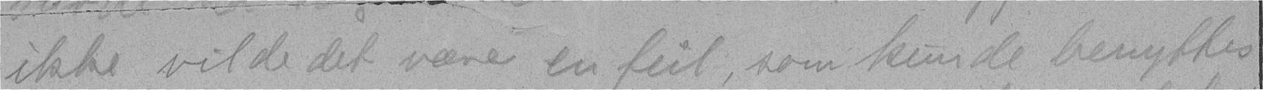ikke vilde det være en feil, som kunde benyttes
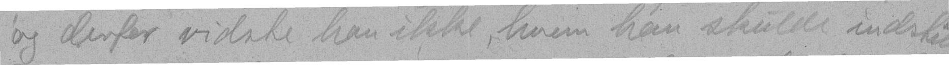og derfor vidste han ikke, hvem han skulde indstille.
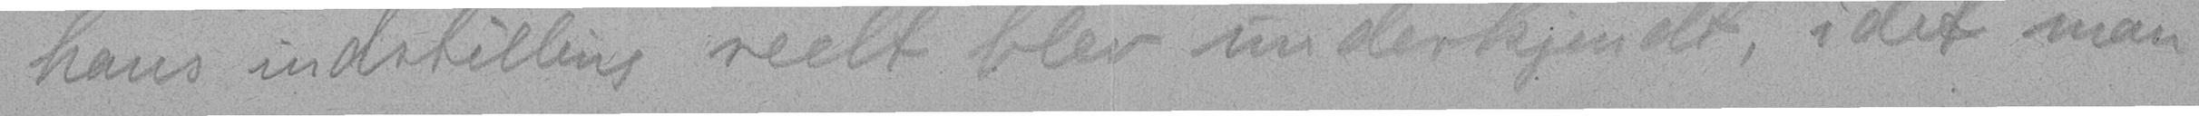hans indstilling reelt blev underkjendt, i det man
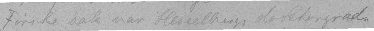Første sak var Hesselbergs doktorgrad.
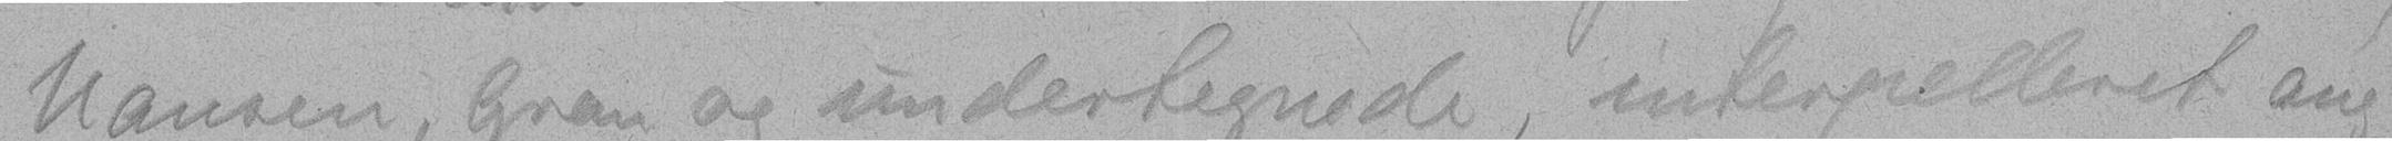Nansen, Gran og undertegnede, interpelleret ang.
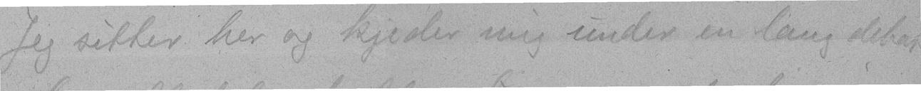Jeg sitter her og kjeder mig under en lang debat
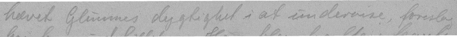hævet Glimmes dygtighet i at undervise, foreslog
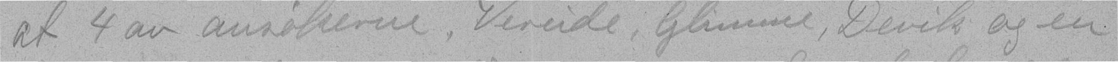at 4 av ansøkerne, Vereide, Glimme, Devik og en
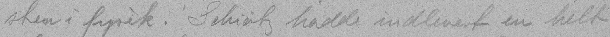sten i fysik. Schiøtz hadde indlevert en helt
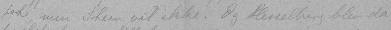1ste, men Steen vil ikke. Og Hesselberg blev da
